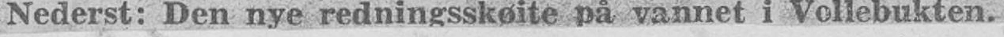Nederst: Den nye redningsskøite på vannet i Vollebukten.
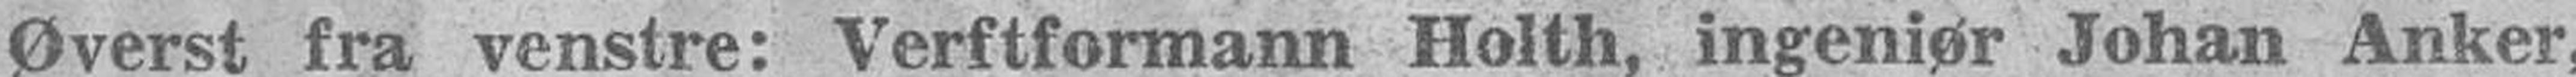Øverst fra venstre: Verftformann Holth, ingeniør Johan Anker,
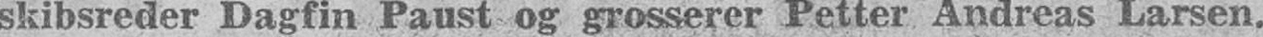skibsreder Dagfin Paust og grosserer Petter Andreas Larsen.
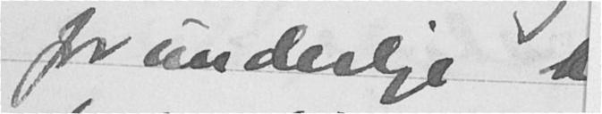forunderlige s
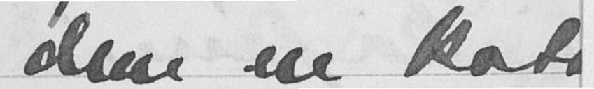dem en kat
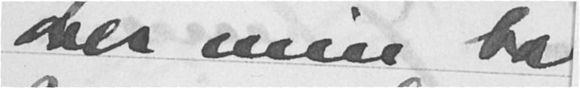over min ba
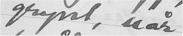grynt, når
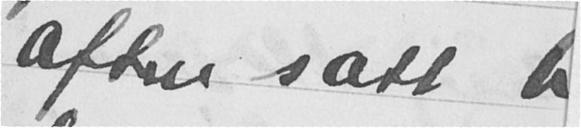aften satt b
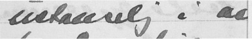ustanselig i a
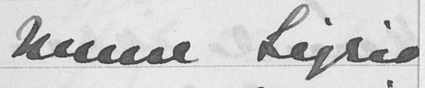kunne Sigrid
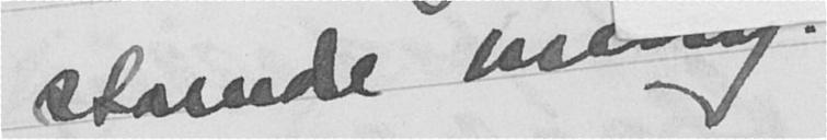stornde userig.
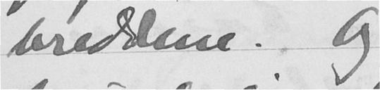breddene. Og
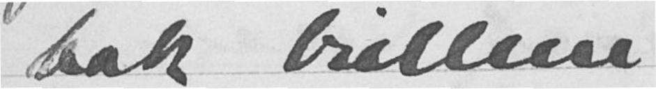bak brillene
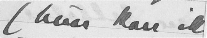(hun kan ik
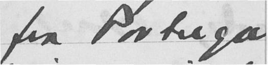fra Portuga
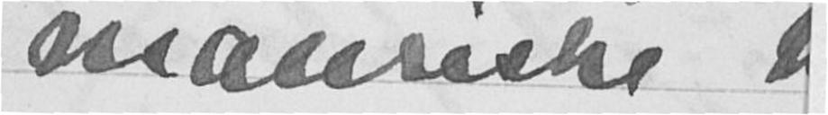mauriske
